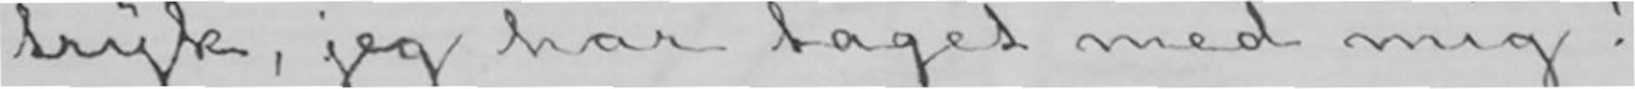tryk, jeg har taget med mig!
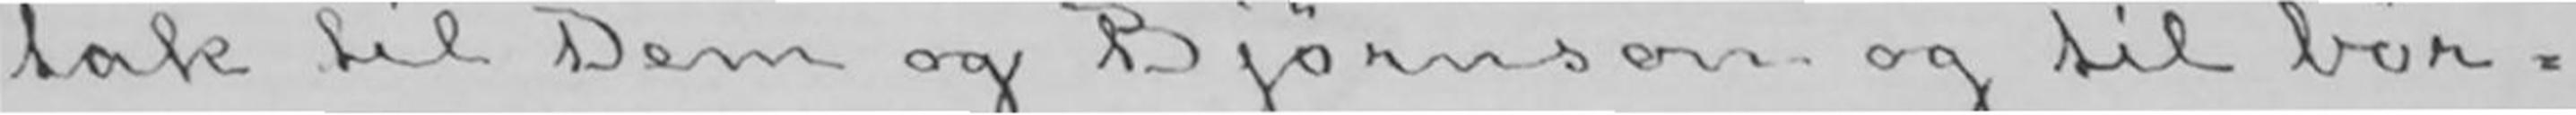tak til Dem og Bjørnson og til bør-
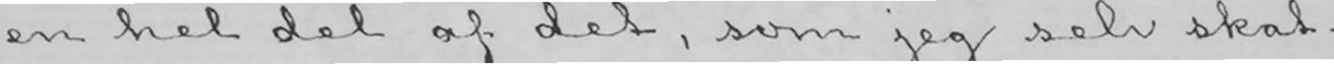en hel del af det, som jeg selv skat-
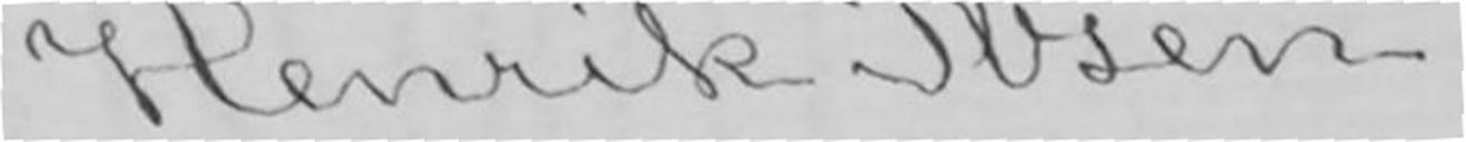Henrik Ibsen.
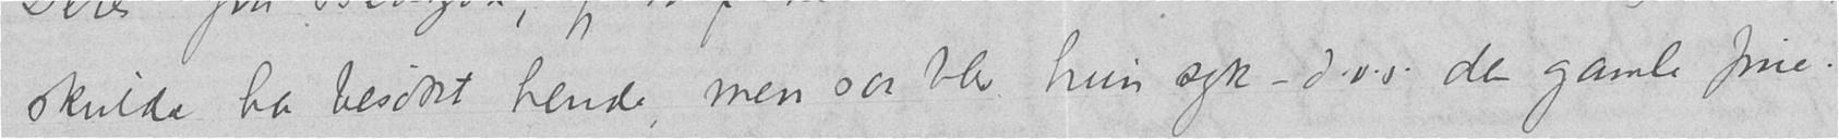skulde ha besøkt hende, men saa blev hun syk – d.v.s. den gamle frue.
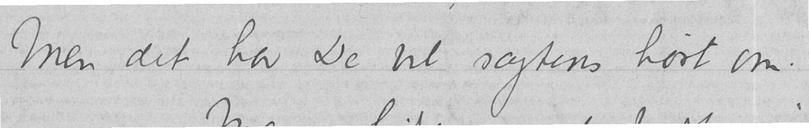Men det har De vel sagtens hørt om.
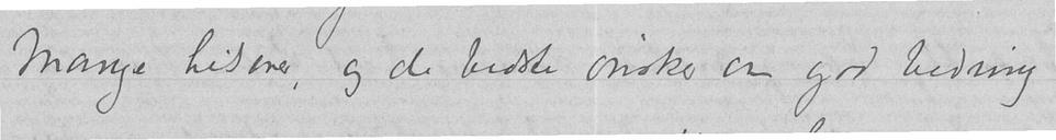Mange hilsener, og de bedste ønsker om god bedring
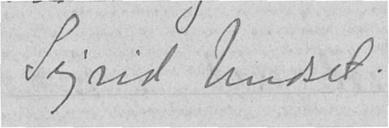Sigrid Undset.
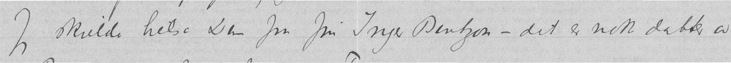Jeg skulde hilse Dem fra fru Inger Bentzon – det er nok datter av
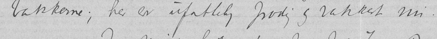bakkerne; her er ufattelig frodig og vakkert nu.
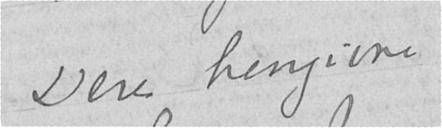Dere hengivne
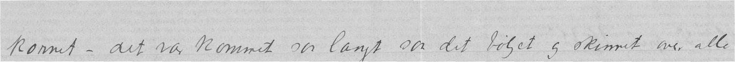kornet – det var kommet saa langt saa det bølget og skinnet over alle
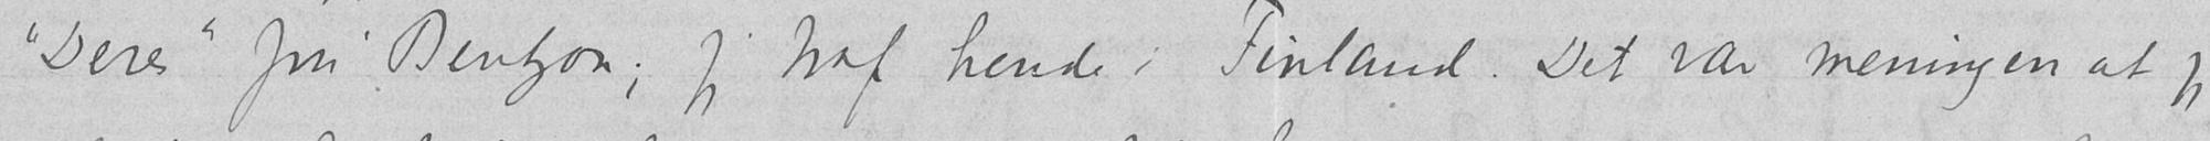"Deres" fru Bentzon; Jeg traf hende i Finland. Det var meningen at jeg
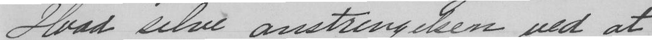Hvad selve anstrengelsen ved at
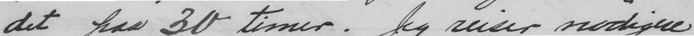det paa 30 timer. Jeg reiser nødigere
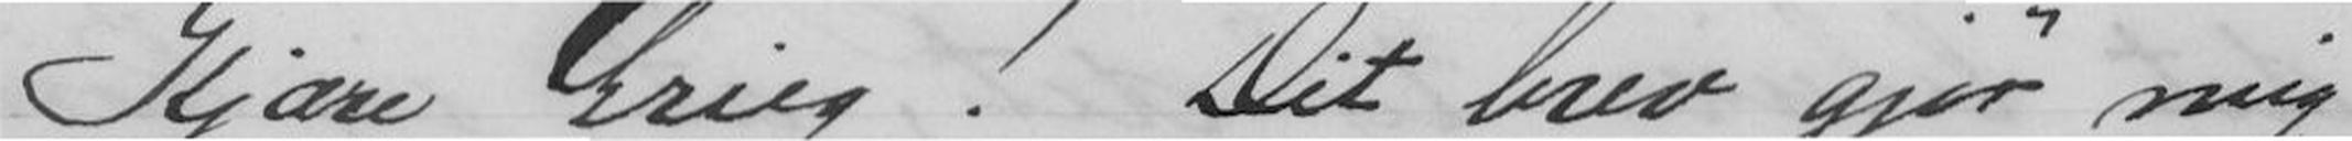Kjære Grieg! Dit brev gjør mig
